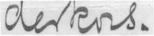derkors.
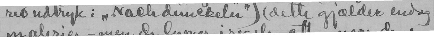res udtryk i "Nachdunckeln") (dette gjælder endog
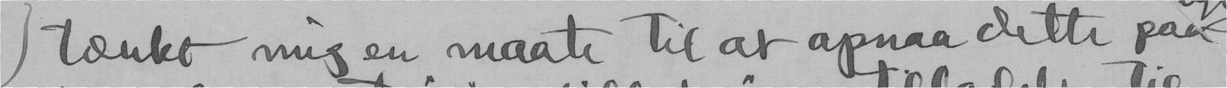tænkt mig en maate til at opnaa dette paa
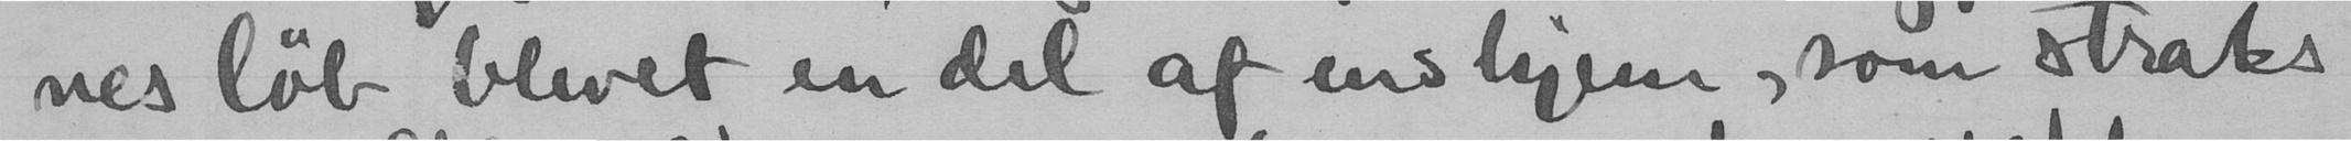nes løb blevet en del af ens hjem, som straks
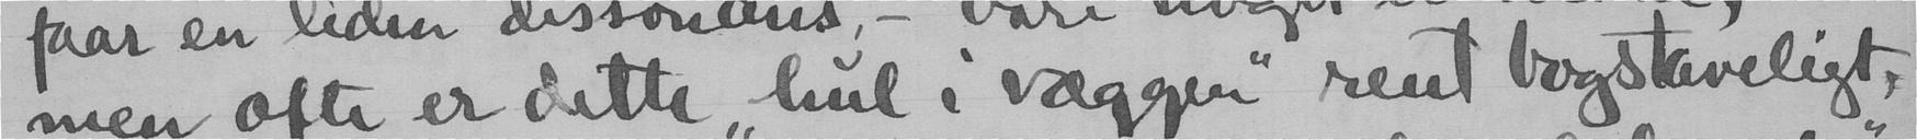men ofte er dette "hul i væggen" rent bogstaveligt,
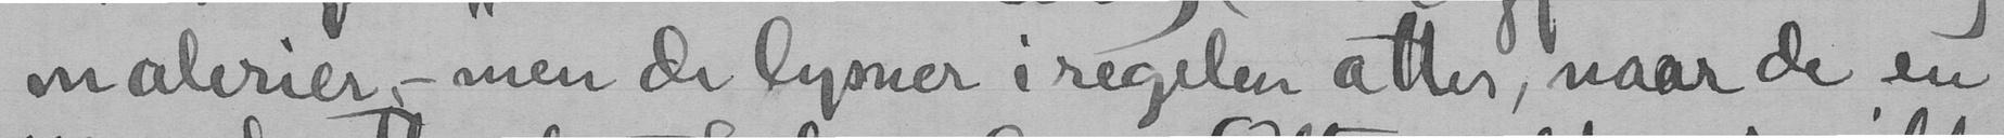malerier - men de lysner i regelen atter, naar de en
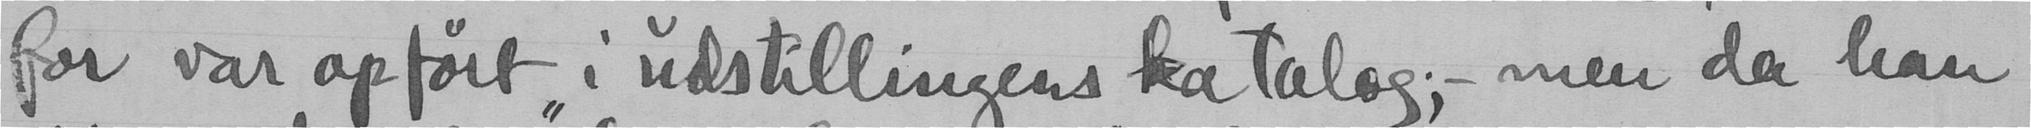for var opført i udstillingens katalog, - men da han
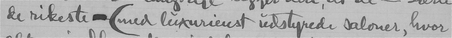de rikeste - (med luxurienst udstyrede saloner, hvor
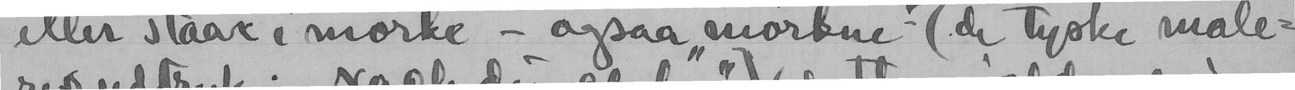eller staar i mørke - ogsaa "mørkne" (de tyske male-
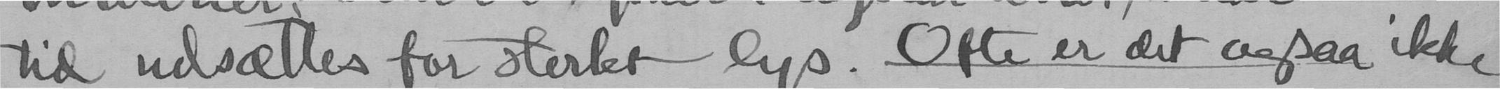tid udsættes for sterkt lys. Ofte er det ogsaa ikke
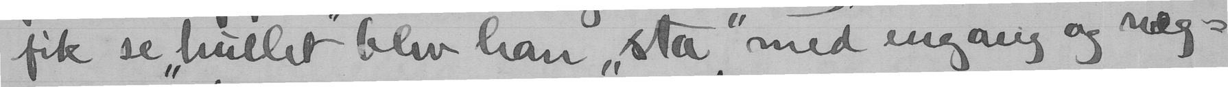fik se "hullet" blev han "sta" med engang og næg-
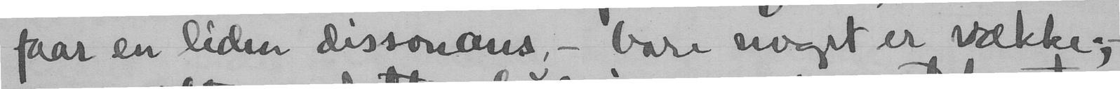faar en liden dissonans, - bare noget er vække; -
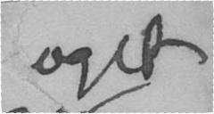og et
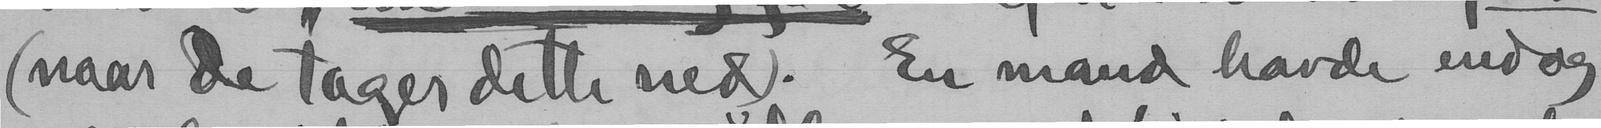(naar de tager dette ned). En mand havde endog
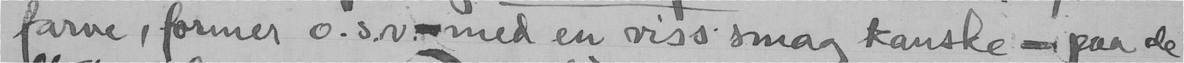farve, former o.s.v. med en viss smag kanske - paa de
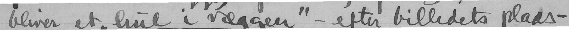bliver et "hul i væggen" - efter billedets plads-
